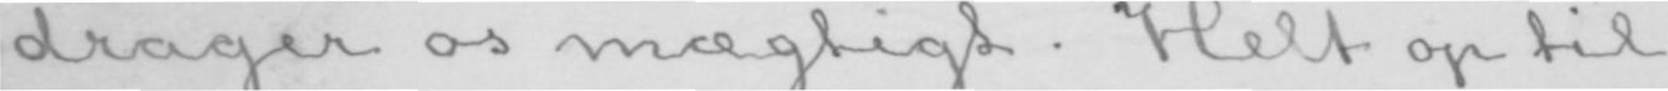drager os mægtigt. Helt op til
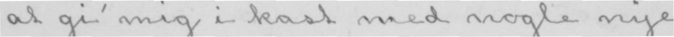at gi&rapo; mig i kast med nogle nye
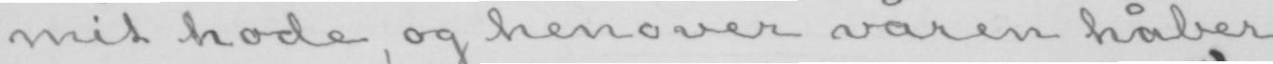mit hode, og henover våren håber
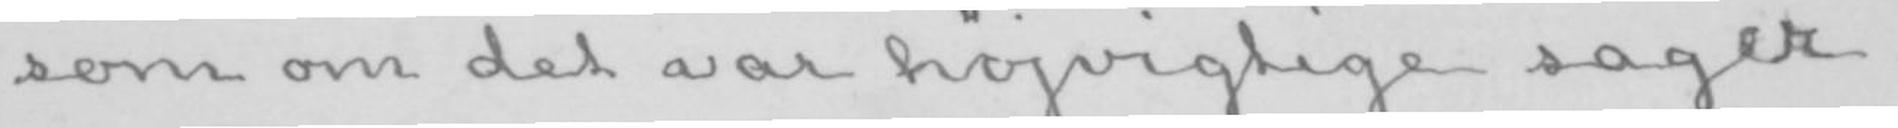som om det var højvigtige sager.
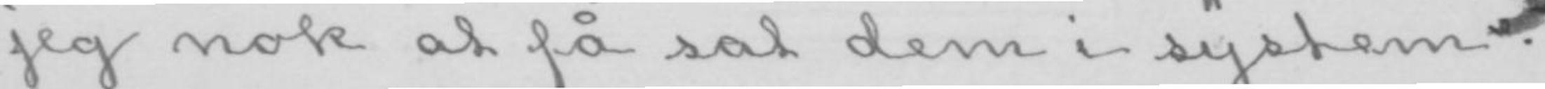jeg nok at få sat dem i system.
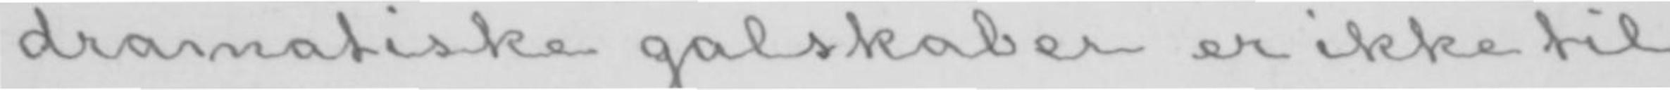dramatiske galskaber er ikke til
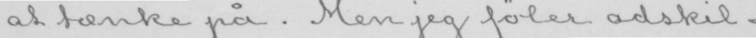at tænke på. Men jeg føler adskil-
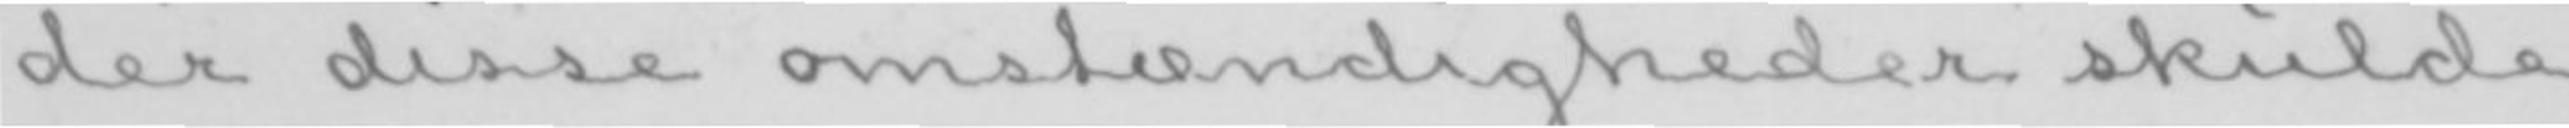der disse omstændigheder skulde
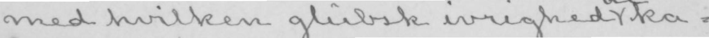med hvilken glubsk ivrighed de ka-
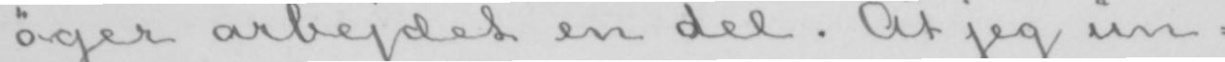øger arbejdet en del. At jeg un-
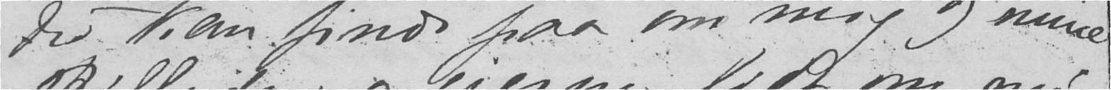du kan finde paa om mig og mine
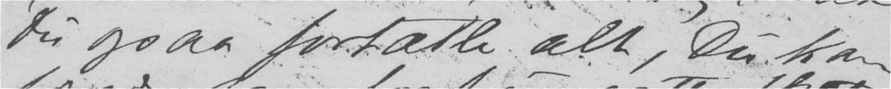du ogsaa fortælle alt, Du kan
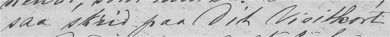saa skriv paa Dit Visitkort
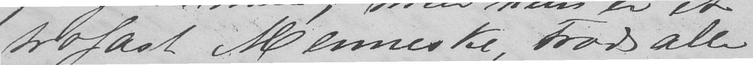trofast Menneske, trods alle
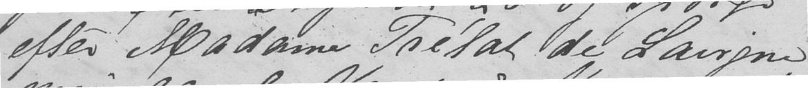efter Madame Trélat de Lavigne,
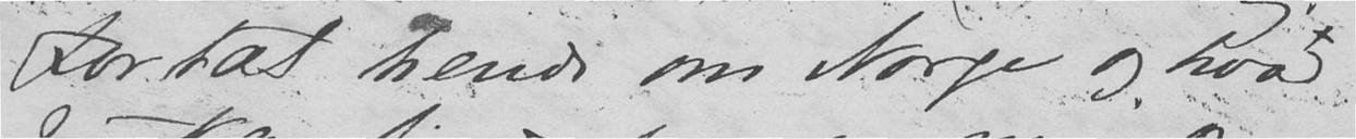Fortæl hende om Norge og hvad
